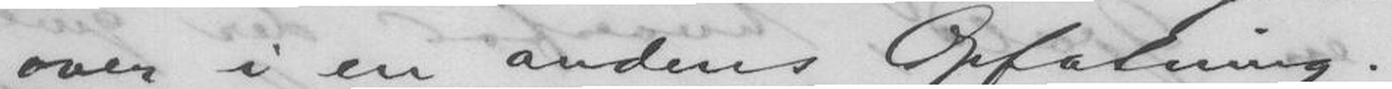over i en andens Opfatning.
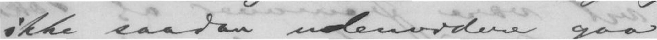ikke saadan udenvidere gaa
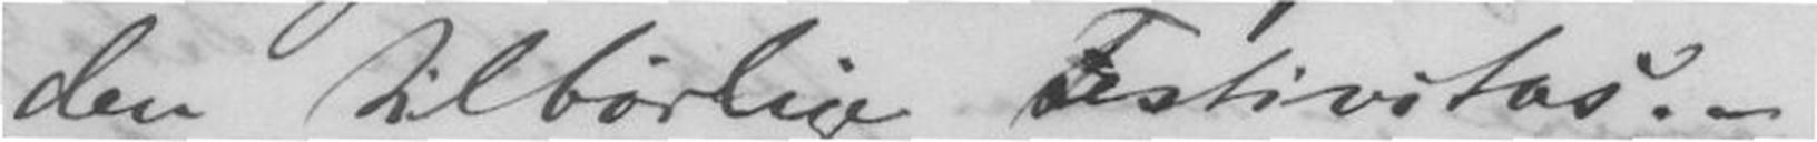den tilbørlige Festivitas. -
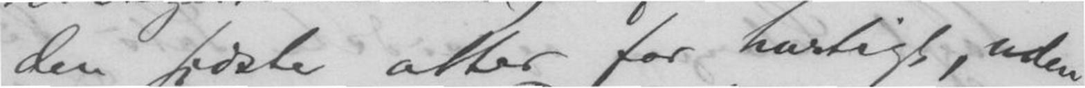den sidste atter for hurtigt, uden
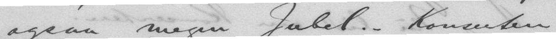ogsaa megen Jubel. Konserten
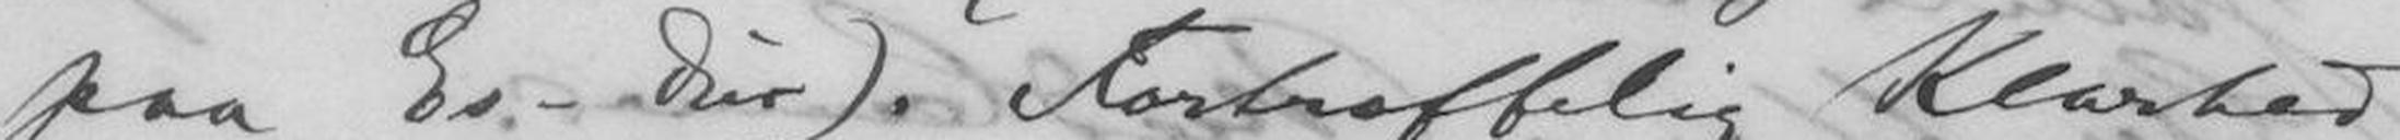paa Es.-Dur). Fortræffelig Klarhed
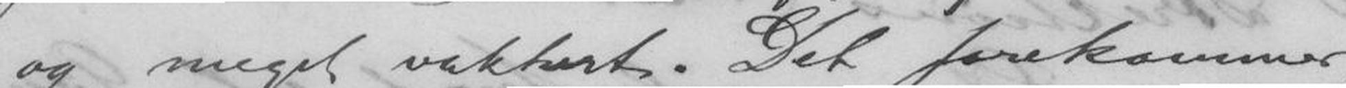og meget vakkert. Det forekommer
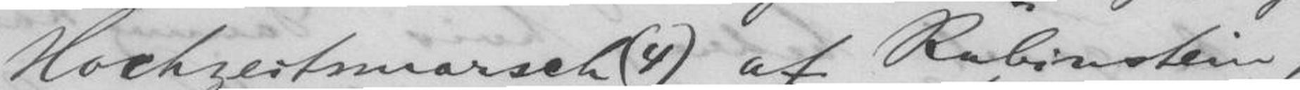Hochzeitsmarsch (4) af Rubinstein,
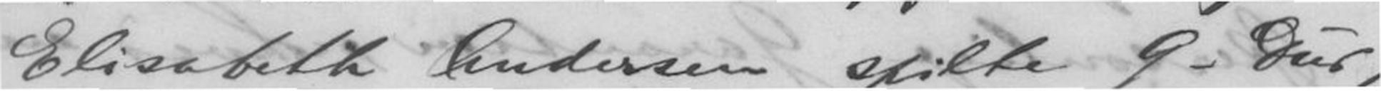Elisabeth Andersen spilte G-Dur,
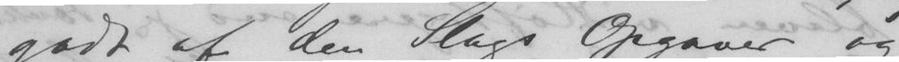godt af den Slags Opgaver og
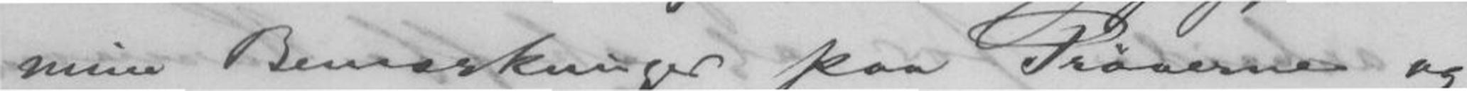mine Bemærkninger paa Prøverne og
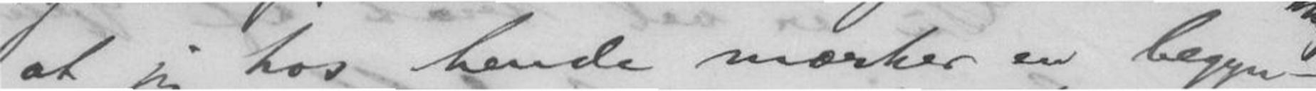at jeg hos hende mærker en begyn-
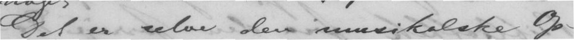Det er selve den musikalske Op-
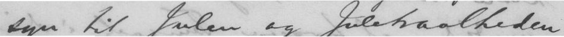syn til Julen og Juletravlheden
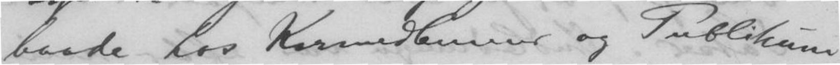baade hos Kormedlemmer og Publikum
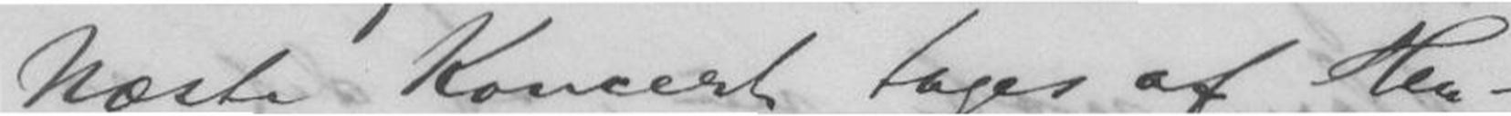Næste Koncert tages af Hen-
